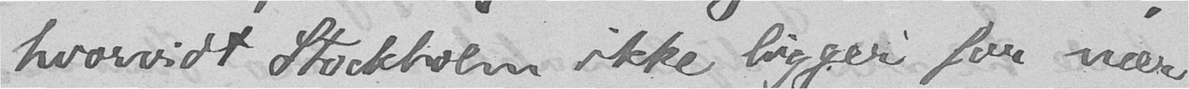hvorvidt Stockholm ikke ligger for nær
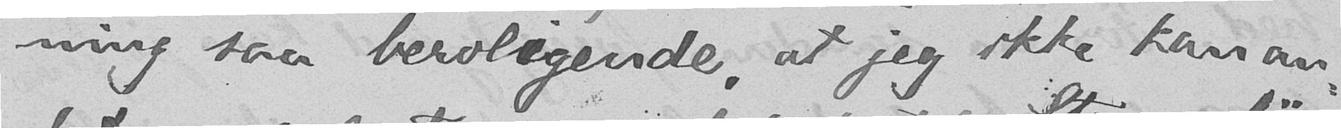ning saa beroligende, at jeg ikke kan an-
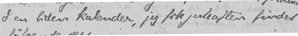I en liden kalender, jeg fik juleaften findes
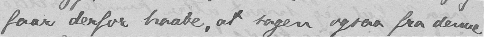faar derfor haabe, at sagen ogsaa fra denne
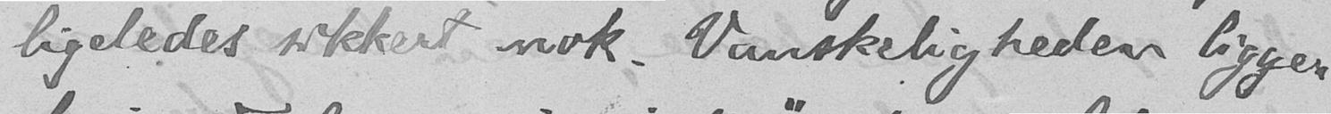ligeledes sikkert nok. Vanskeligheden ligger
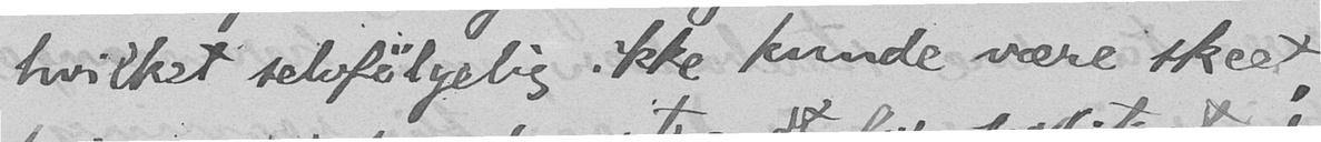hvilket selvfølgelig ikke kunde være skeet
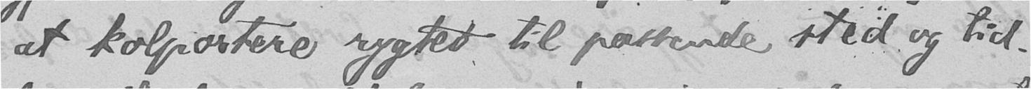at kolportere rygtet til passende sted og tid.
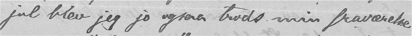jul blev jeg jo ogsaa trods min fraværelse
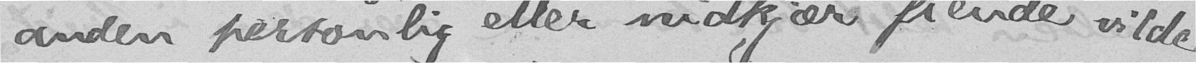anden personlig eller nidkjær fiende vilde
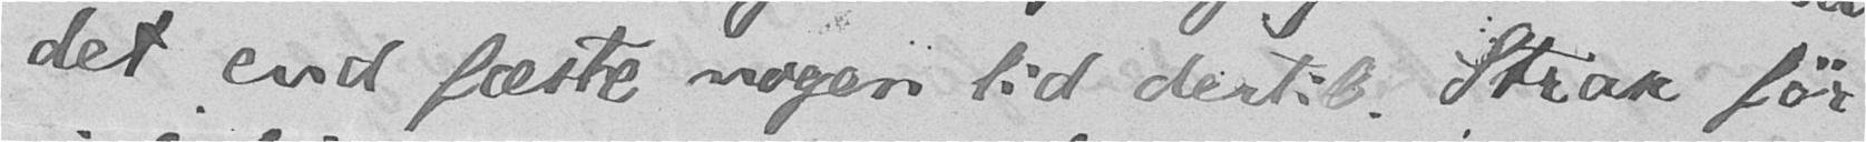det end fæste nogen lid dertil. Strax før
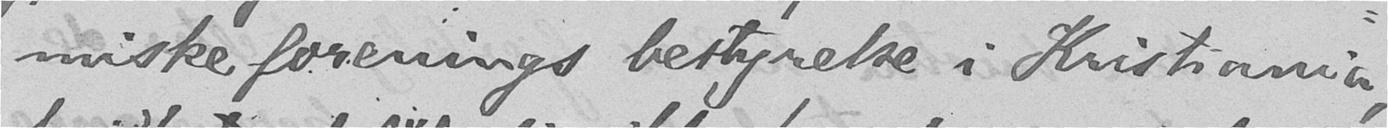miske forenings bestyrelse i Kristiania,
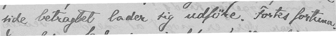side betragtet lader sig udføre. Fortes fortuna.
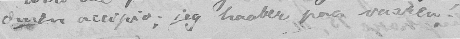Omen accipio; jeg haaber paa vacken!
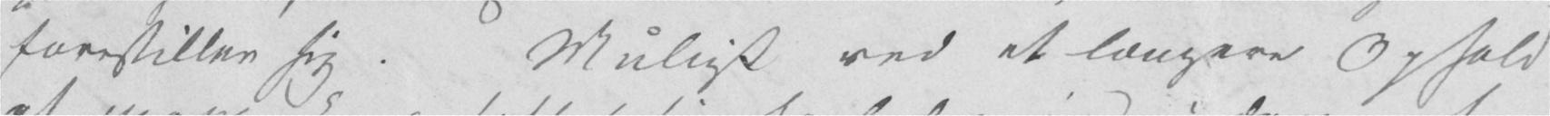forestiller sig. Muligt ved et længere Ophold
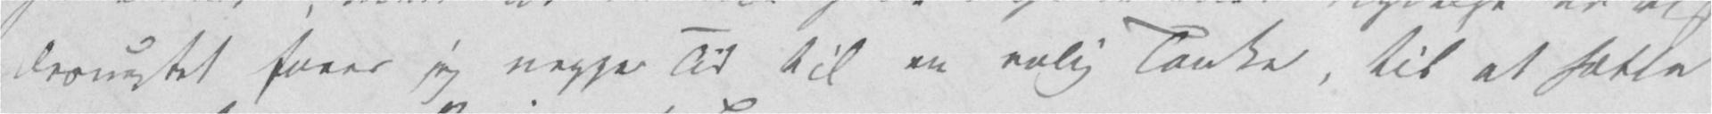desuagtet faaer jeg neppe Tid til en rolig Tanke, til at sætte
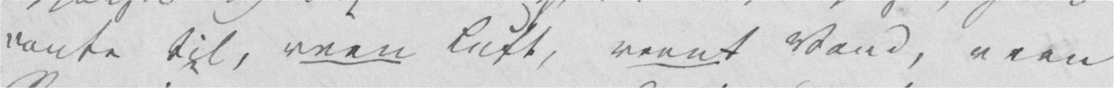vante til, reen Luft, reent Vand, reen
